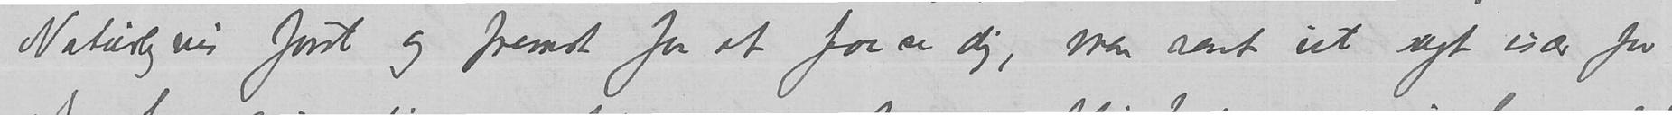Naturligvis først og fremst for at faa se dig, men rent ut sagt især for
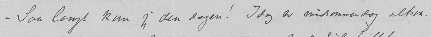Saa langt kom jeg den dagen! Idag er midsommerdag altsaa.
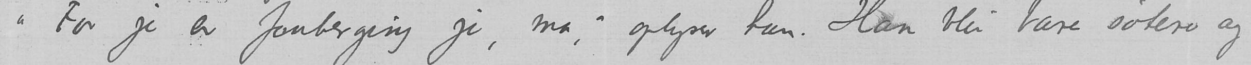i «For je er faaberging je, ma,» oplyser han. Han blir bare søtere og
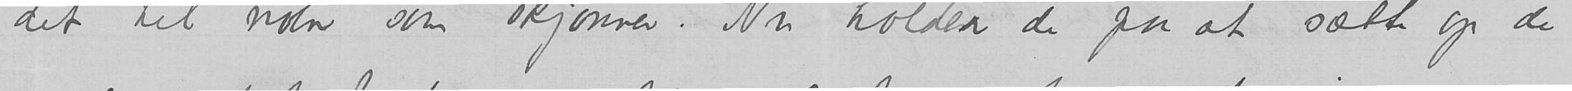det til noen som skjønner. Nu holder de paa at sætte op de
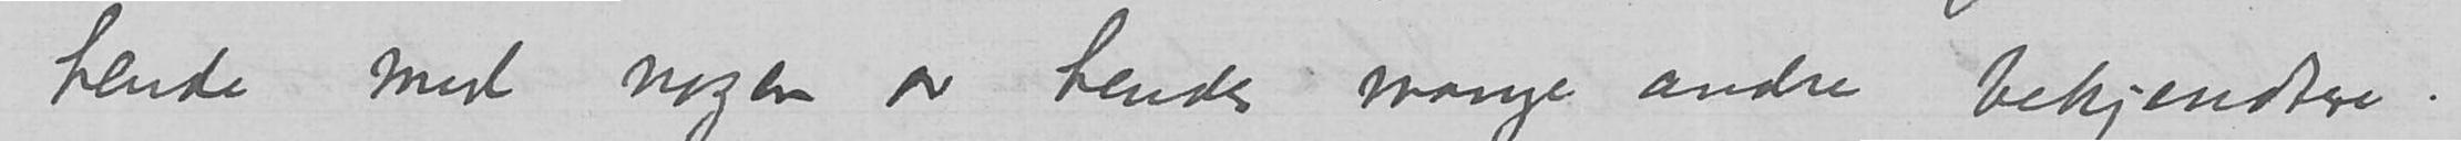hende med nogen av hendes mange andre bekjendtere.
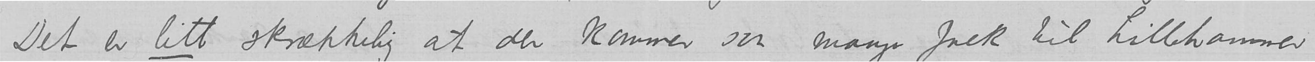Det er litt skrækkelig at der kommer saa mange folk til Lillehammer
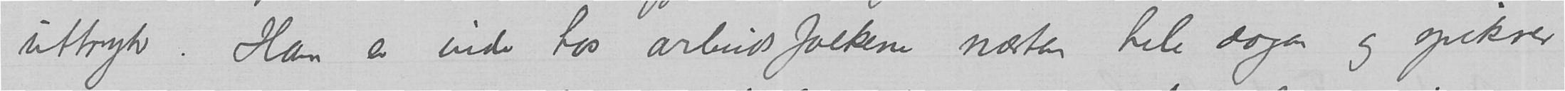uttryk.  Han er inde hos arbeidsfolkene næsten hele dagen og spikrer
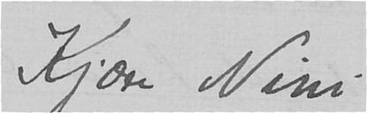Kjære Nini,
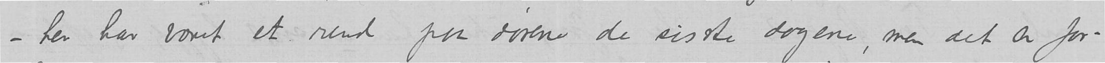– her har været et rend paa dørene de sisste dagene, men det er for-
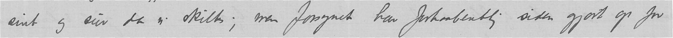sint og sur da vi skiltes; men forsonet har forhaabentlig siden gjort op for
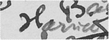Harriet
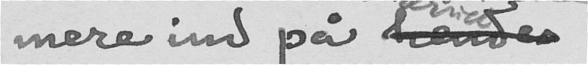mere ind på hendes
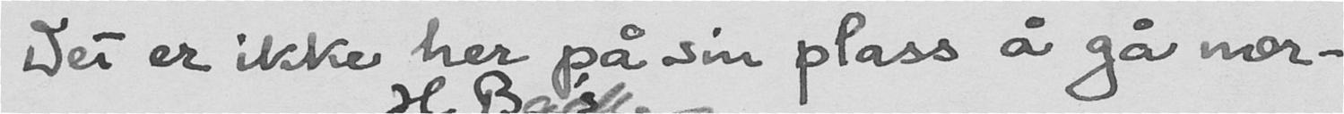Det er ikke her på sin plass å gå nær-
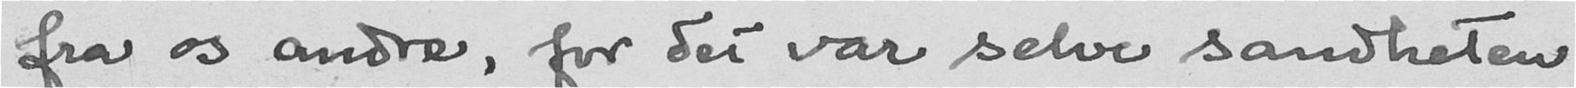fra os andre, for det var selve sandheten
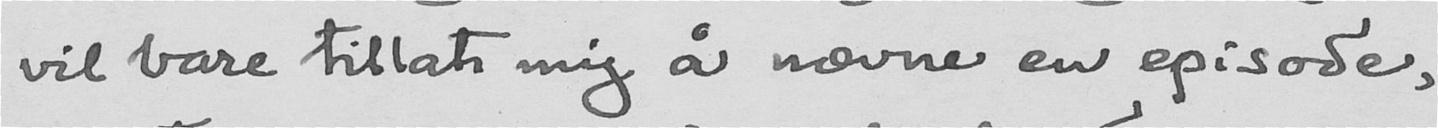vil bare tillate mig å nævne en episode,
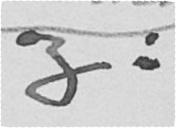og i
